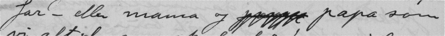far - eller mama og  papa som
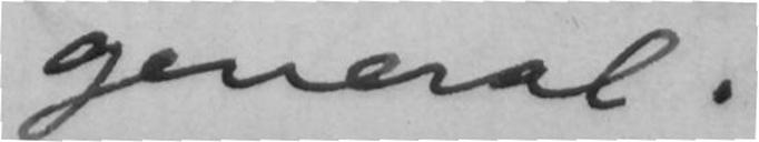general.
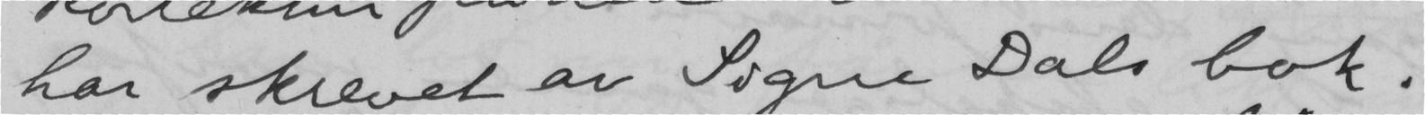har skrevet av Signe Dals bok.
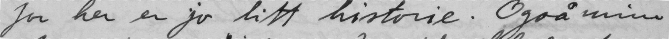for her er jo litt historie. Også mine
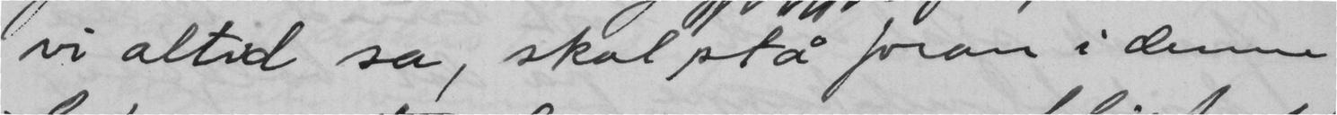vi altid sa, skal stå foran i denne
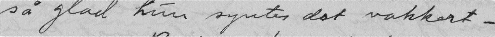så glad hun syntes det vakkert -
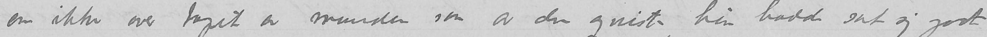om ikke over tapet av manden som av den qvist, hun hadde sat sig godt
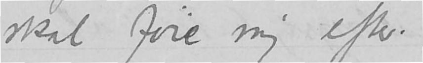føie mig efter.
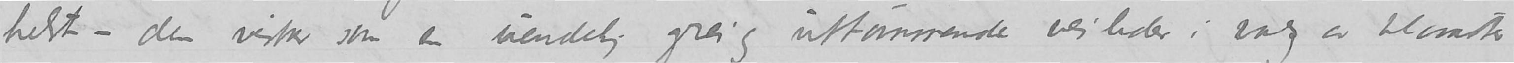helst – den virker som en uendelig grei og uttømmende veileder i brug av blomster
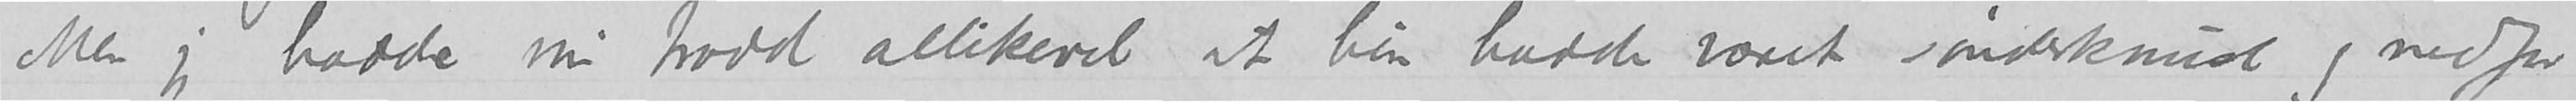Men jeg hadde nu trodd allikevel at hun hadde været sønderknust og nedfor
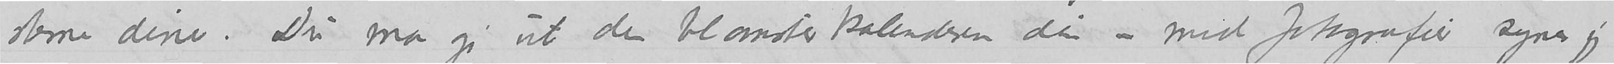sterne dine. Du maa gi ut den blomsterkalenderen din – med fotografier synes jeg
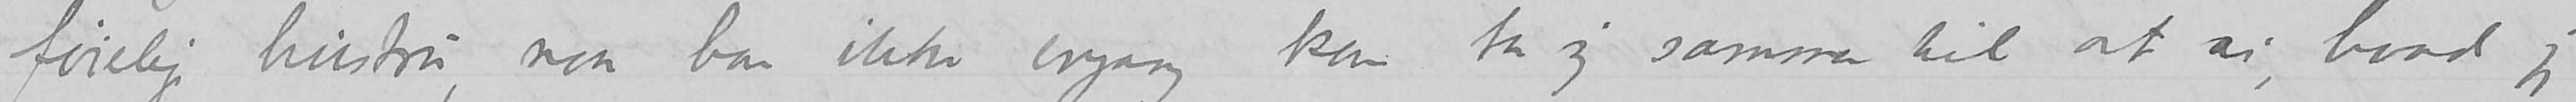føielige hustru, naar han ikke engang kan ta sig sammen til at si, hvad jeg skal
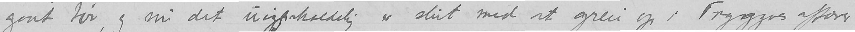gaat tør, og nu det uigjenkaldelig er slut med at greie op i Tryggves affærer
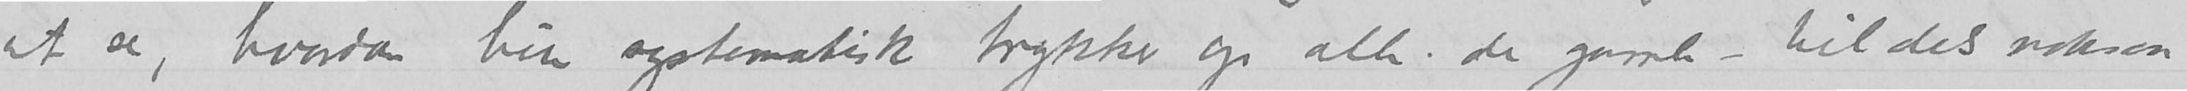hvordan hun systematisk trykker op alle de gamle – til dels noksaa
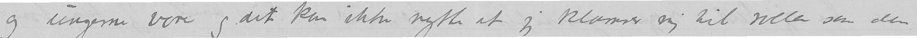og ungerne vore og det kan ikke nytte at jeg klamrer mig til rollen som den
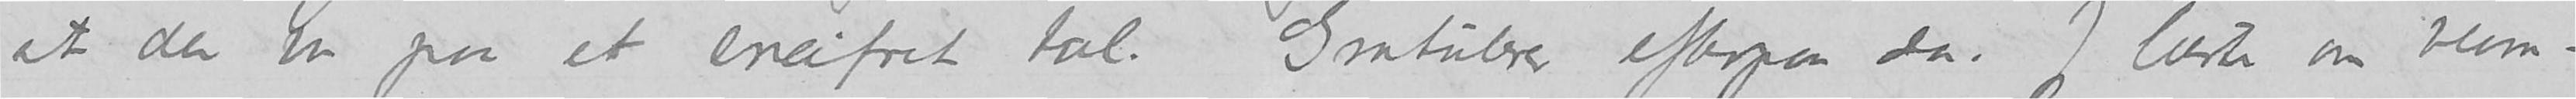at den vaer paa et ensifret tal.  Gratulerer efterpaa da. Jeg læste om blom-
